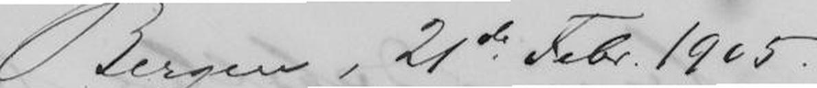Bergen, 21de Febr. 1905.
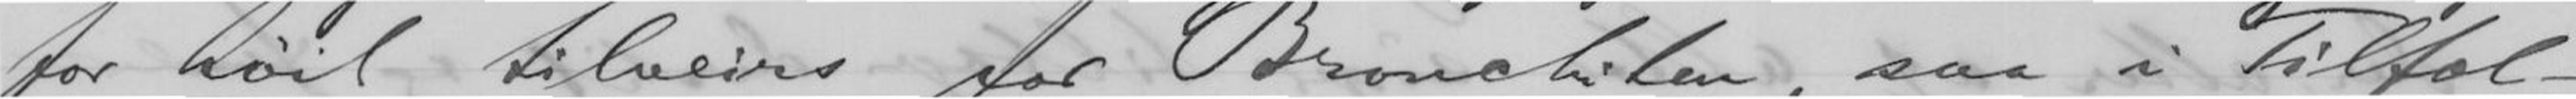for høit tilveirs for Bronchiten, saa i Tilfæl-
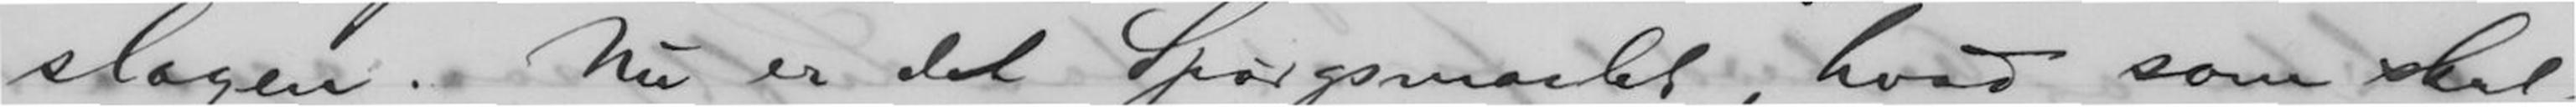slagen. Nu er det Spørgsmaalet, hvad som skul-
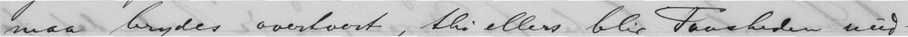maa brydes overtvert, thi ellers blir Tausheden uud-
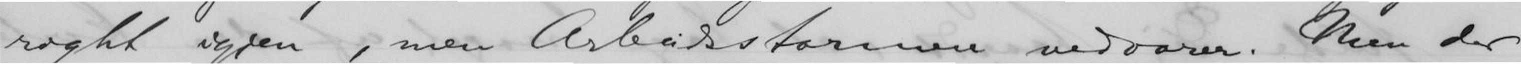right igjen, men Arbeidsstormen vedvarer. Men der
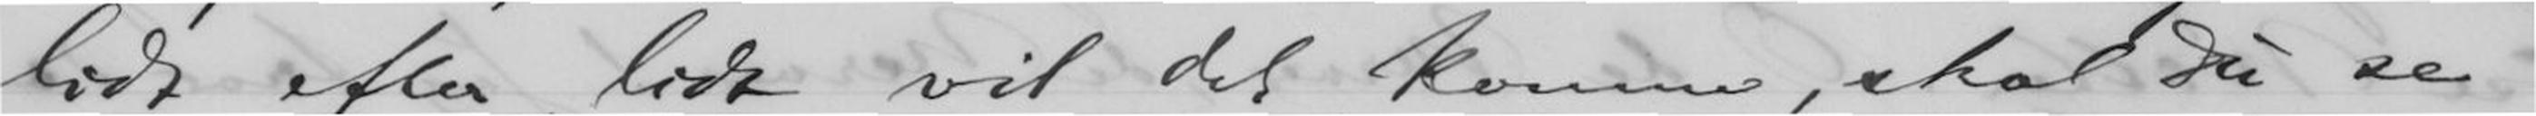lidt efter lidt vil det komme, skal Du se,
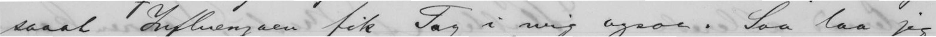saaat Influenzaen fik Tag i mig ogsaa. Saa laa jeg
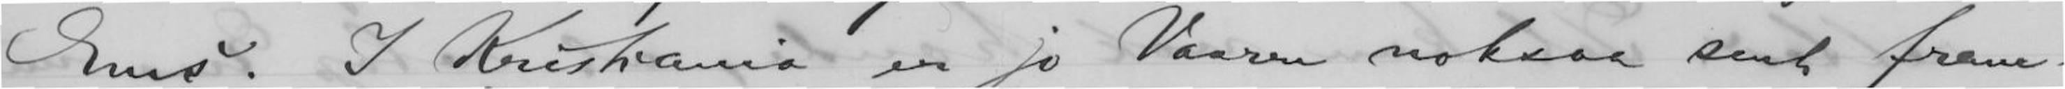Ems. I Kristiania er jo Vaaren noksaa sent frem-
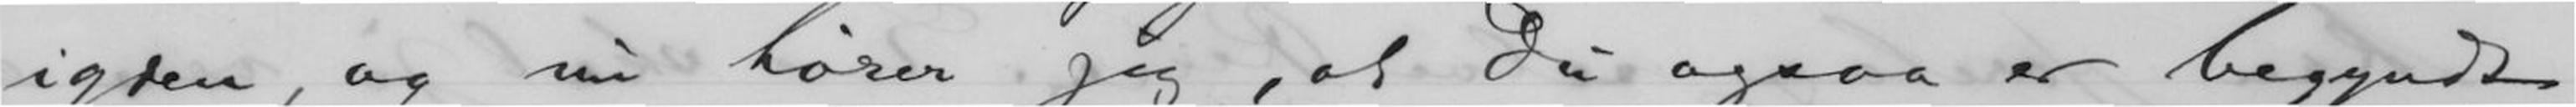igjen, og nu hører jeg, at Du ogsaa er begyndt
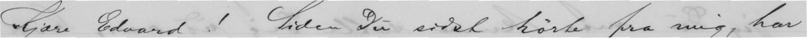Kjære Edvard! Siden Du sidst hørte fra mig, har
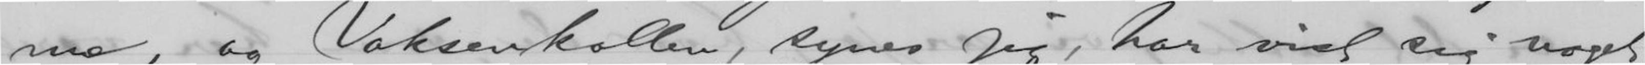me, og Voksenkollen, synes jeg, har vist sig noget
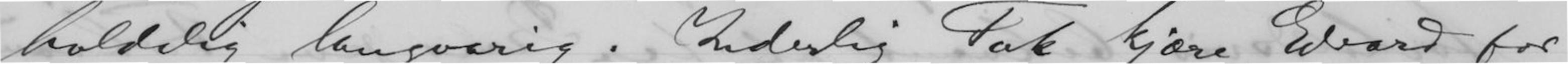holdelig langvarig. Inderlig Tak kjære Edvard for
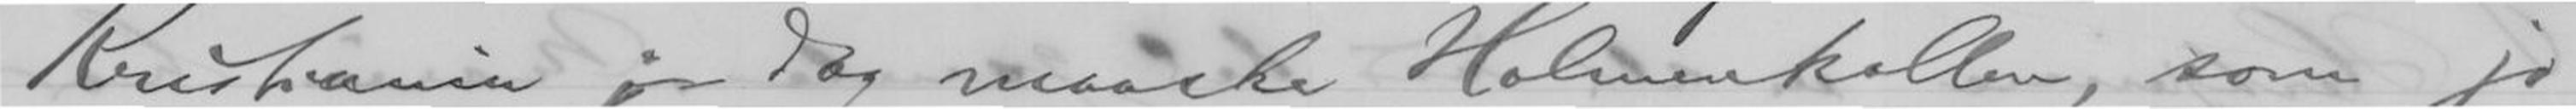Kristiania; dog maaske Holmenkollen, som jo
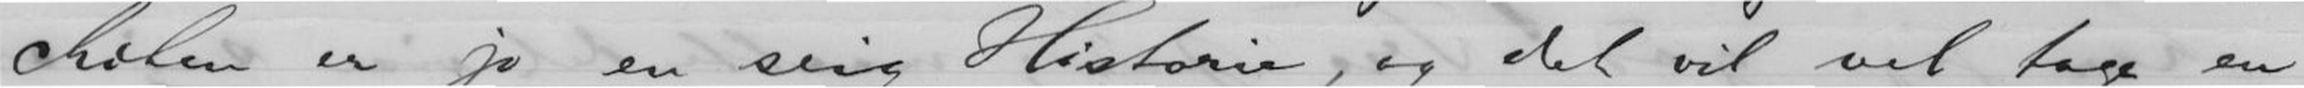chiten er jo en seig Historie, og det vil vel tage en
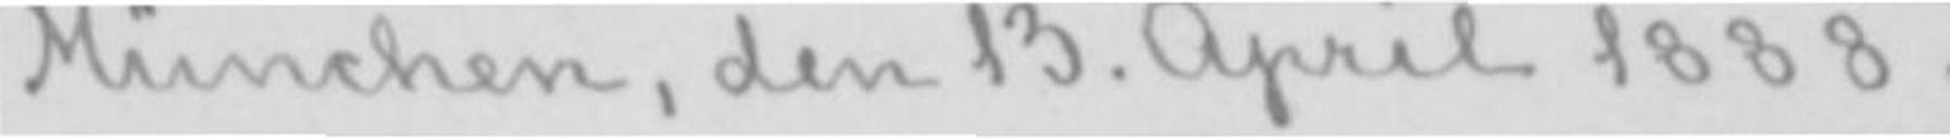München, den 13. April 1888.
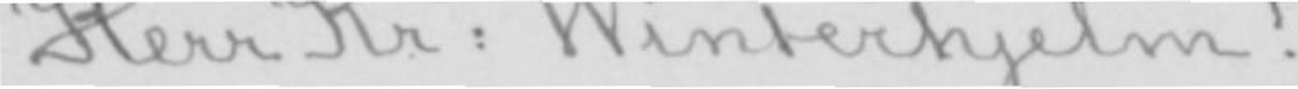Herr Kr: Winterhjelm!
